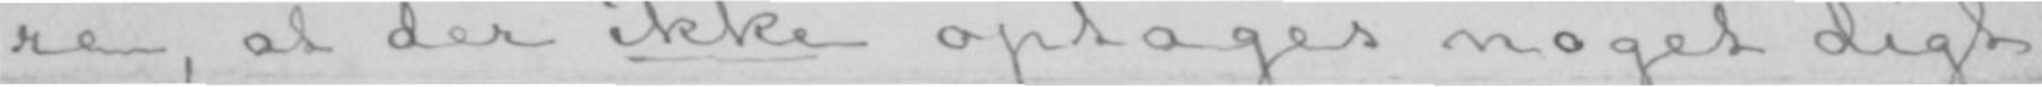re, at der ikke optages noget digt
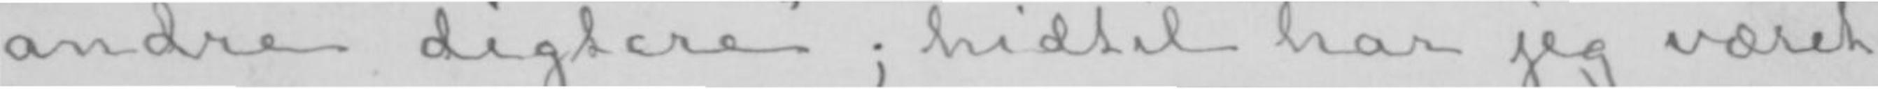«andre digtere»; hidtil har jeg været
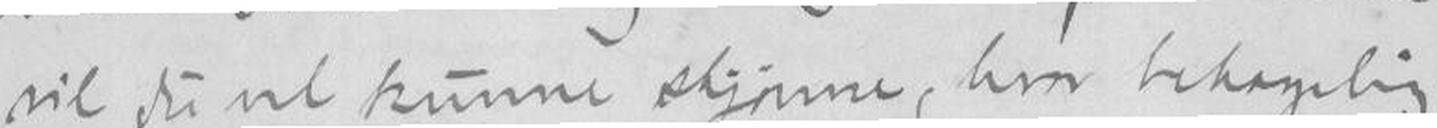vil du vel kunne skjønne, hvor behagelig
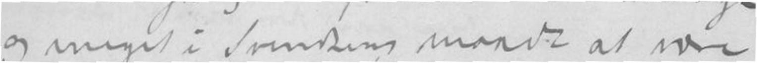og meget i Svendsens maade at være
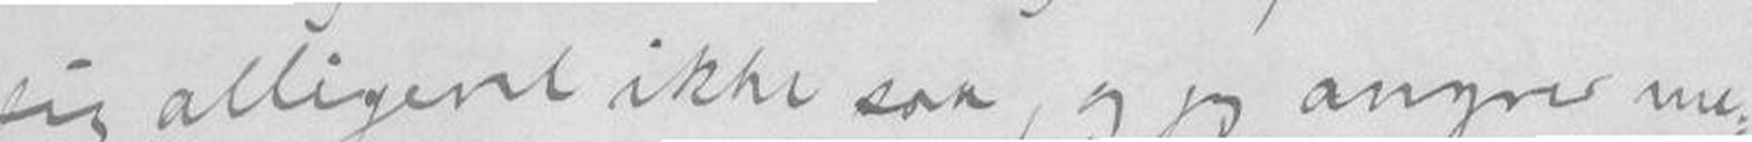sig alligevel ikke saa, og jeg angrer me-
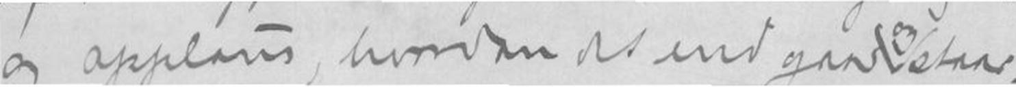og applaus, hvordan det end gaar staar
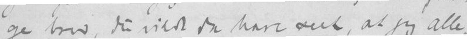ge brev, du vilde da have seet, at jeg alle-
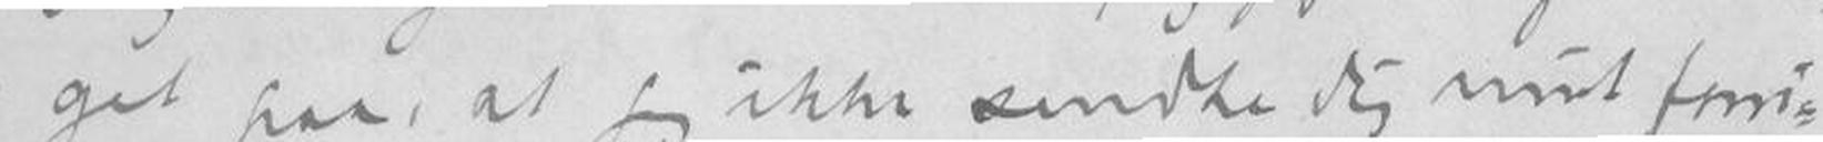get paa, at jeg ikke sendte dig mit forri-
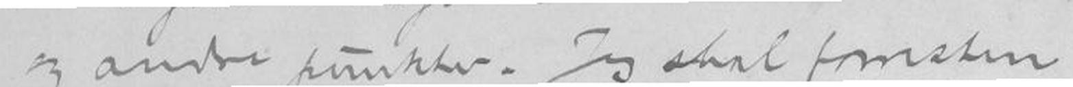og andre punkter. Jeg skal forresten
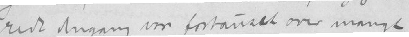rede dengang var forbauset over mangt
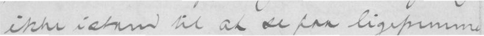ikke istand tie at se paa ligefremme
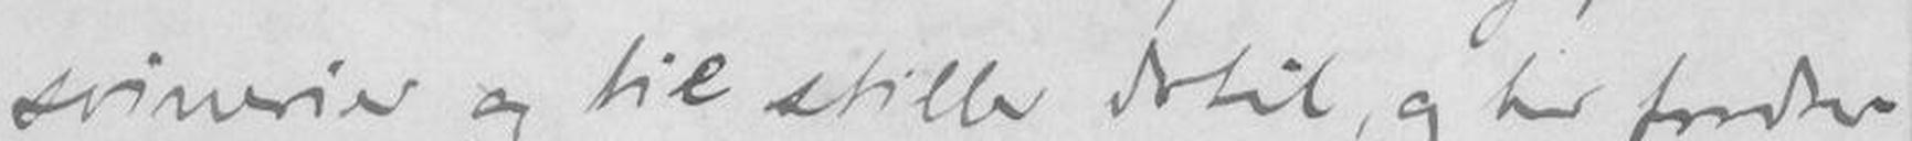svinerier og til stille dertil og han fordrer
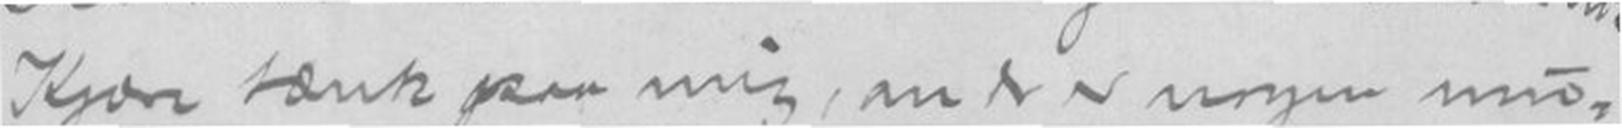Kjære tænk paa mig, om det er nogen mu-
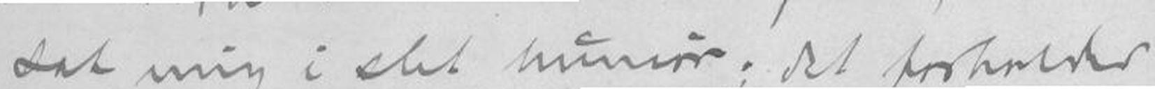sat mig i slet humør; det forholder
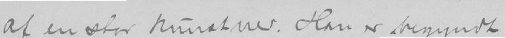af en stor kunstner. Han er begyndt
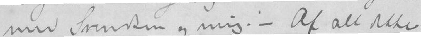med Svendsen og mig.- Af alt dette
